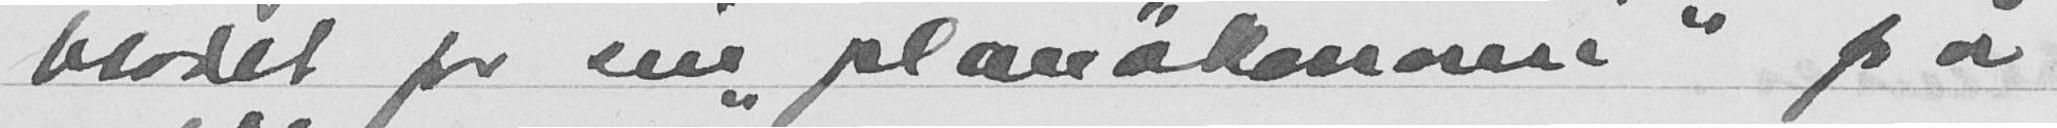bladet for sin "planøkonomi" på
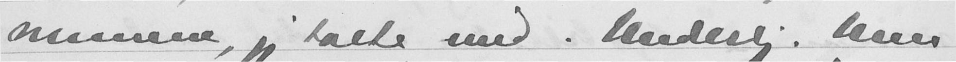mennene, jeg talte med. Underlig. Men
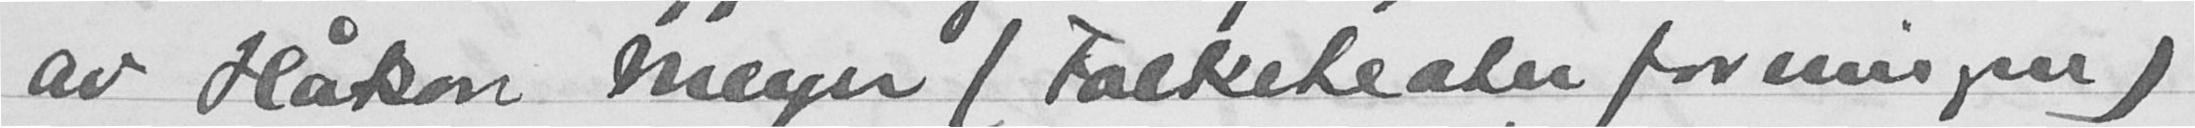av Håkon Meyer (Folketeaterforeningen)
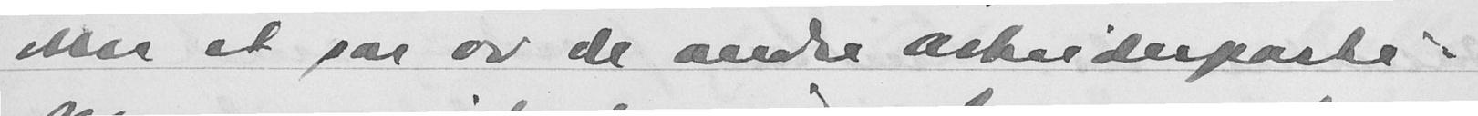eller et par av de andre arbeiderparti-
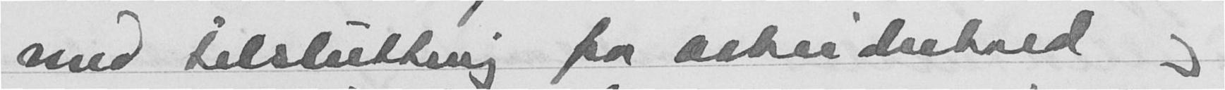med tilslutting fra arbeiderhold og
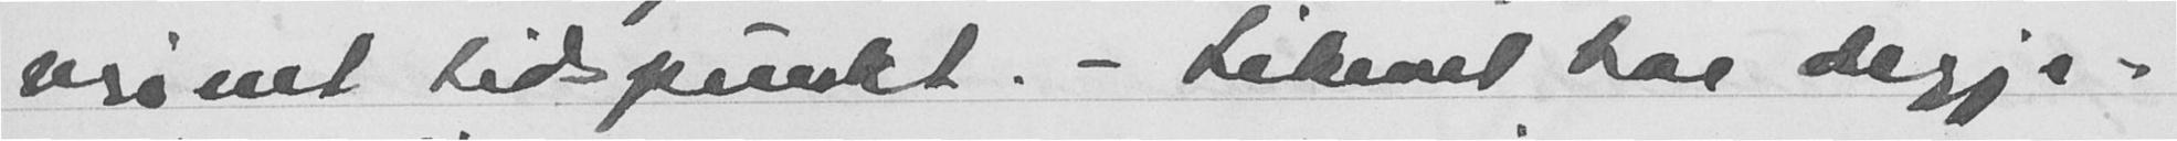signet tidspunkt. - Likevel har regje-
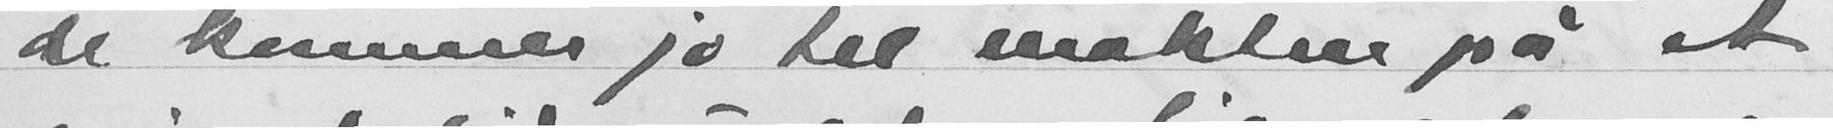de komme jo til makten på et
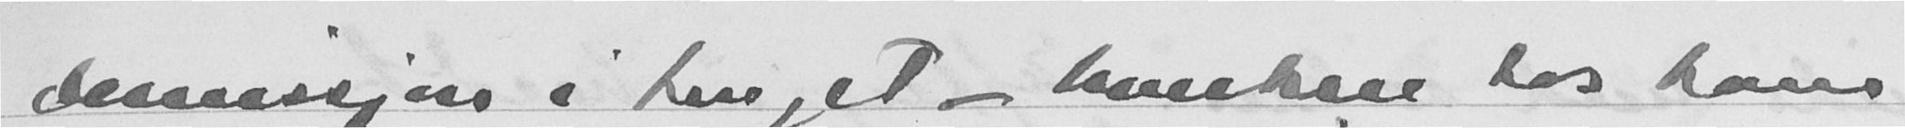desveissjøn i tinget - bunkere hos kan
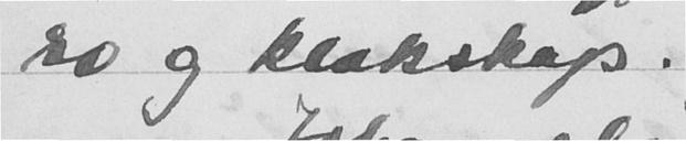ro og klokskap.
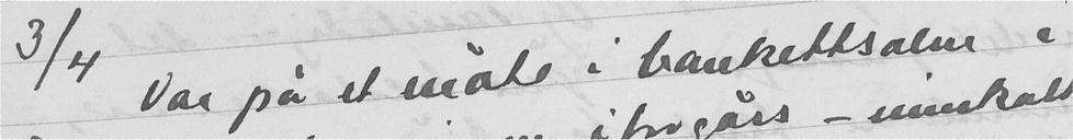3/4 Var på et møte i bankettsalen i
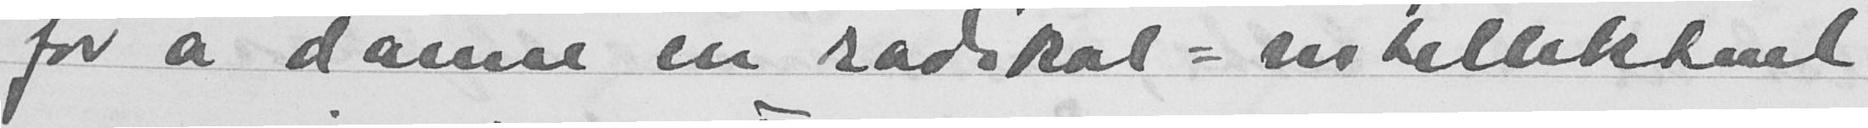for å danne en radikal-intellektuel
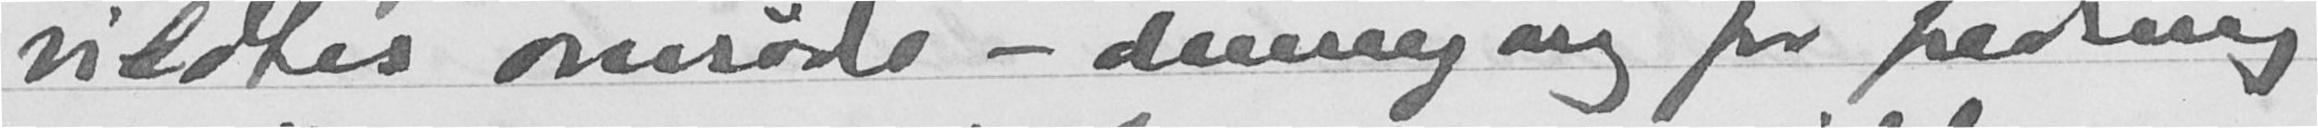vildtes område - dennegang for fredning
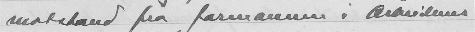motstand fra formannen i Arbeidernes
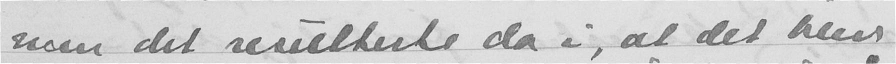men det resulterte da i, at det blir
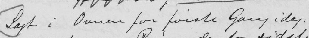Lagt i Ovnen for første Gang idag.
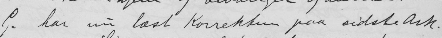G. har nu læst Korektur paa sidste Ark.
